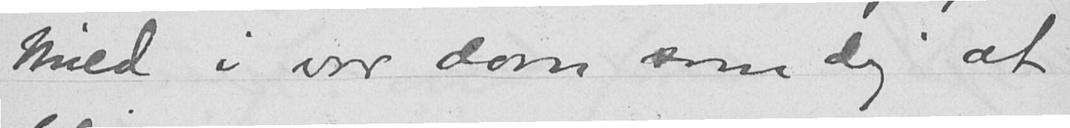Mild i vor dom som dig at
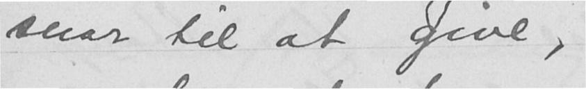snar til at give,
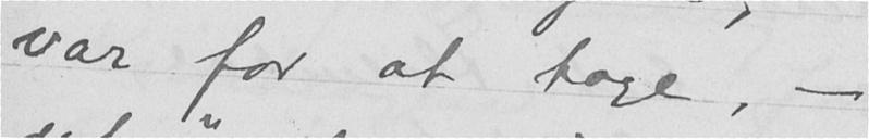var for at tage, -
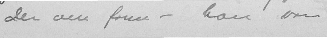der om form - han var
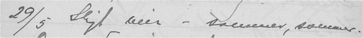29/5 Sligt veir - sommer, sommer.
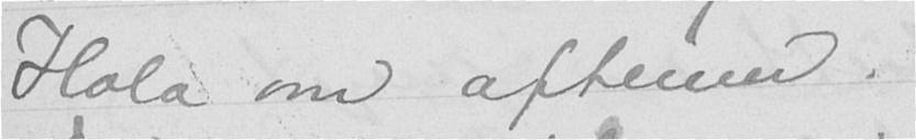Hola om aftenen.
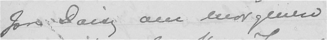Jon Darig om morgenen
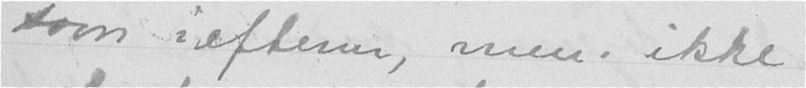søvn i efterm, men ikke
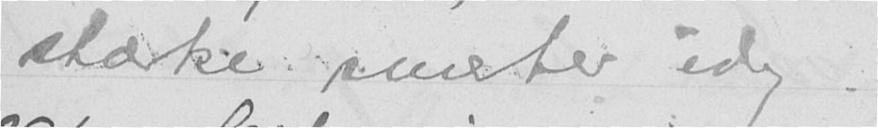stærke smerter idag.
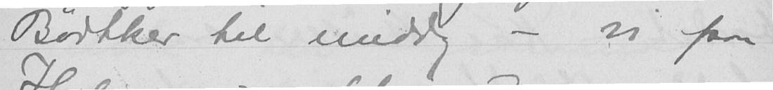Bødtker til middag - vi paa
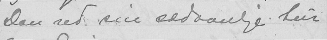Don red sin sædvanlige tur
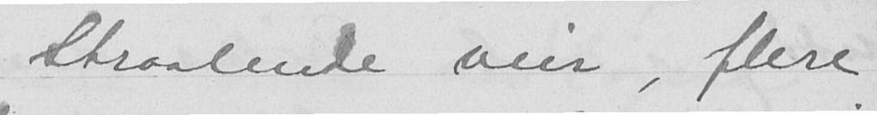Straalende veir, flere
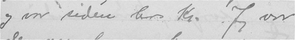og var siden hos Kr. Jeg var
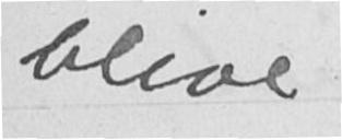blive
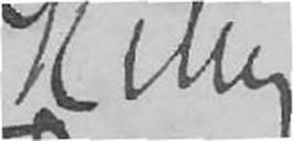Kitty
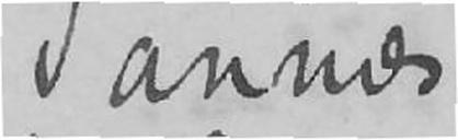Tannæs
